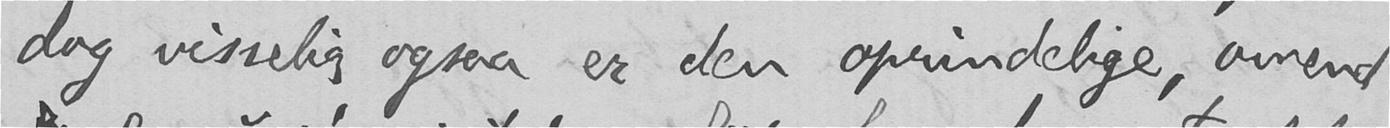dog visselig ogsaa er den oprindelige, omend
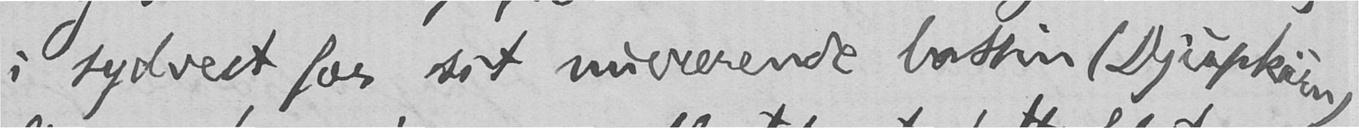i sydvest for sit nuværende bassin (Djupkärn).
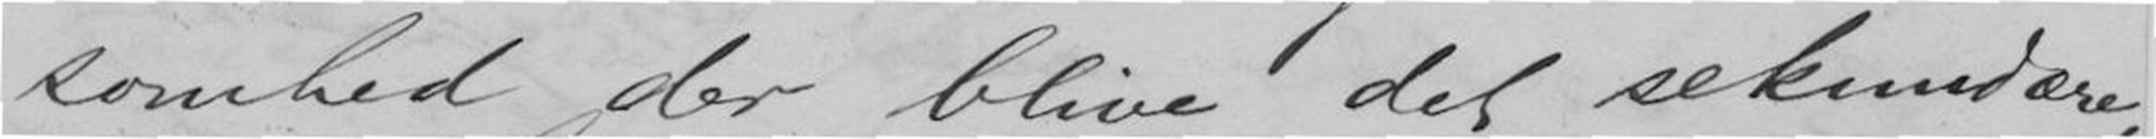somhed der blive det sekundære,
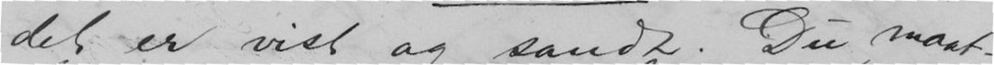det er vist og sandt. Du maat-
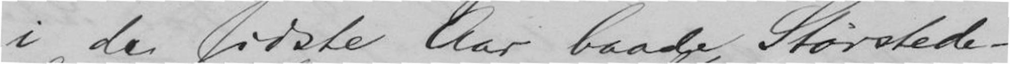i de sidste Aar baade Størstede-
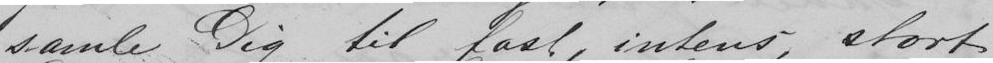samle Dig til fast, intens, stort
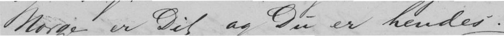Norge er Dit og Du er hendes.
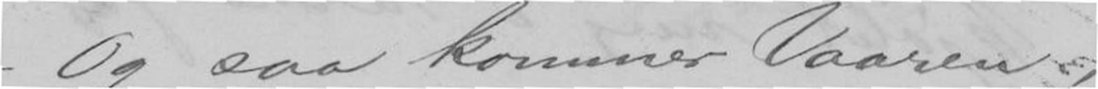Og saa kommer Vaaren,
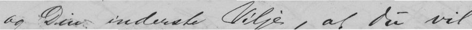og Din inderste Vilje, at du vil
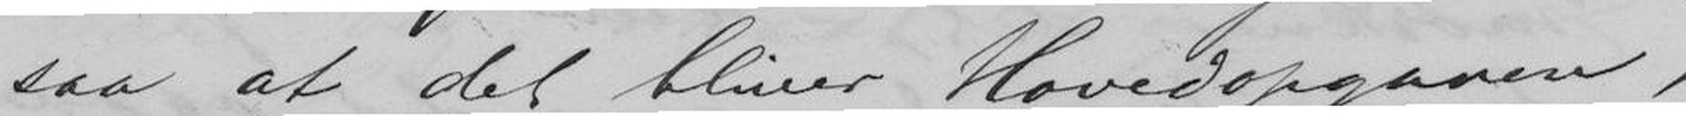saa at det bliver Hovedopgaven,
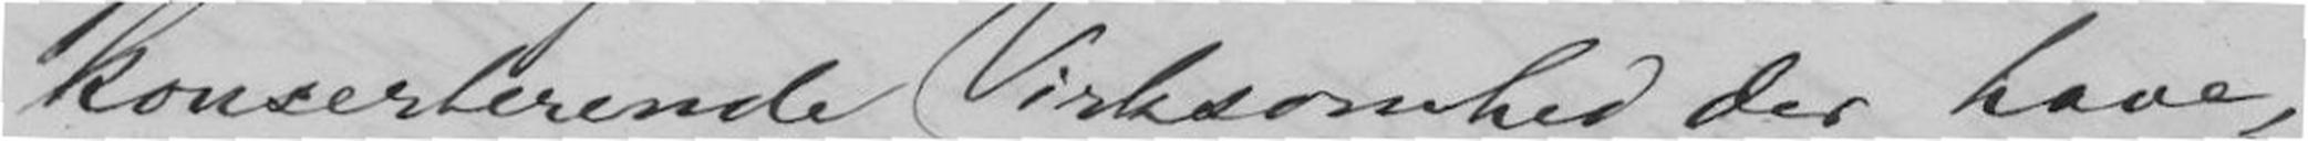konserterende Virksomhed der have,
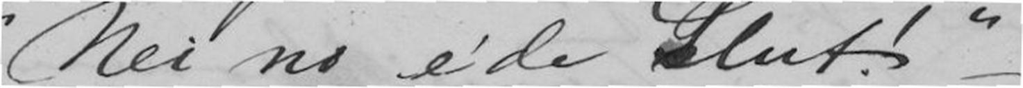"Nei no e'de Slut."
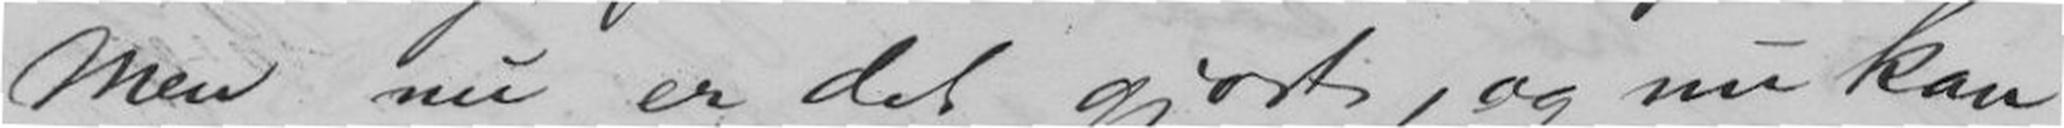Men nu er det gjort, og nu kan
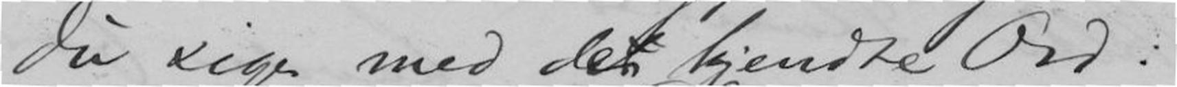du sige med det kjendte Ord:
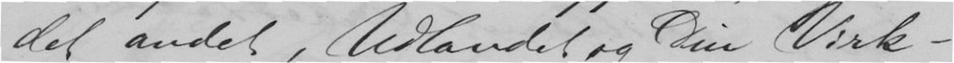det andet, Udlandet og Din Virk-
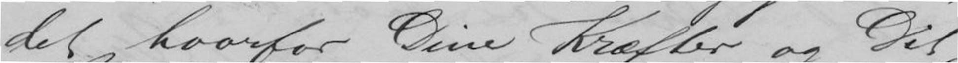det hvorfor Dine Kræfter og Dit
